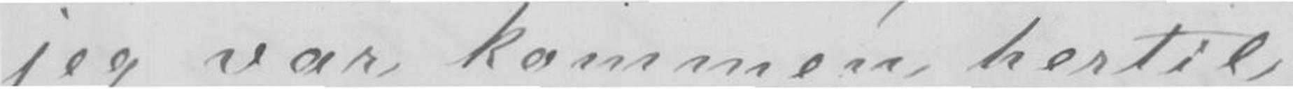jeg var kommen hertil,
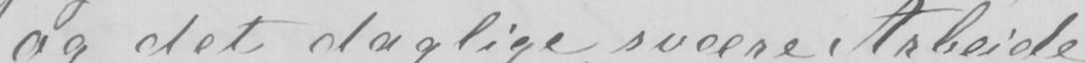og det daglige, svære Arbeide
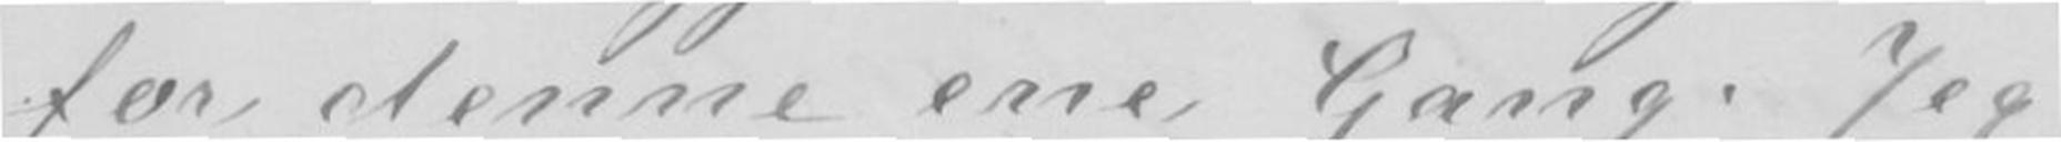for denne ene Gang. Jeg
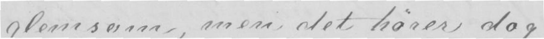glemsom, men det hører dog
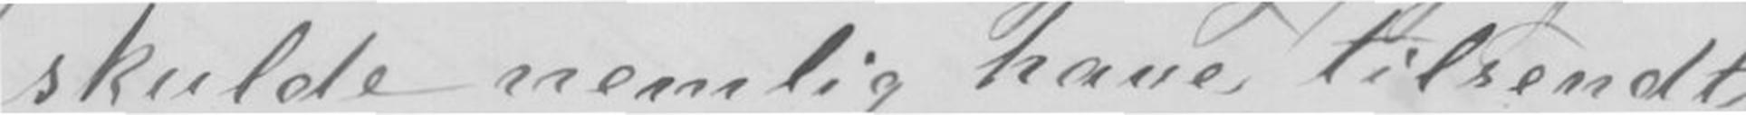skulde nemlig have tilsendt
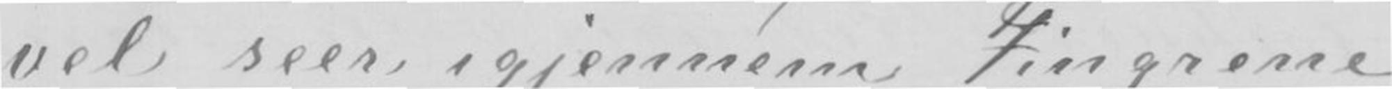vel seer igjennem Fingrene
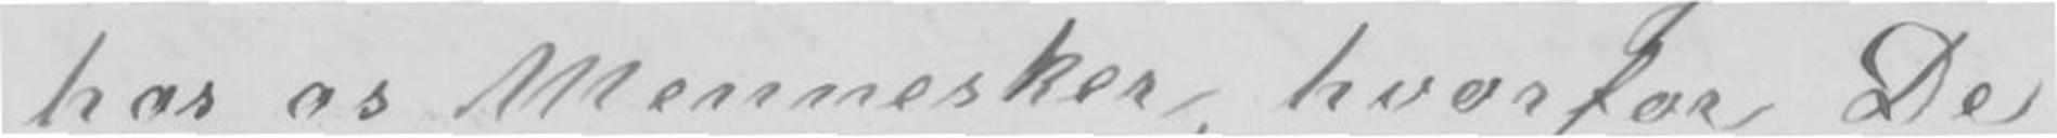hos os Mennesker, hvorfor De
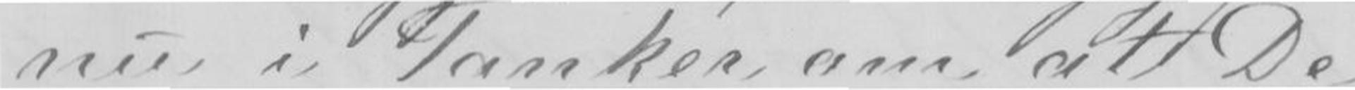nu i Tanker om, at De,
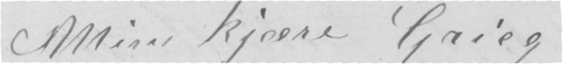Min kjære Grieg
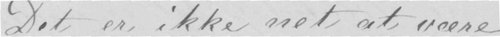Det er ikke net at være
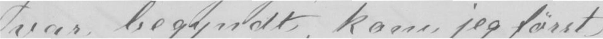var begyndt, kom jeg først
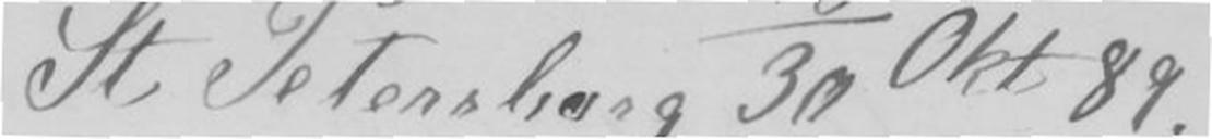St Petersburg 18/30 Okt 89.
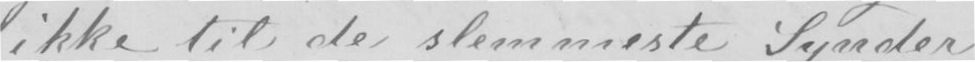ikke til de slemmeste Synder
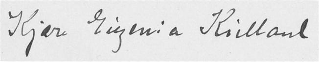Kjære Eugenia Kielland
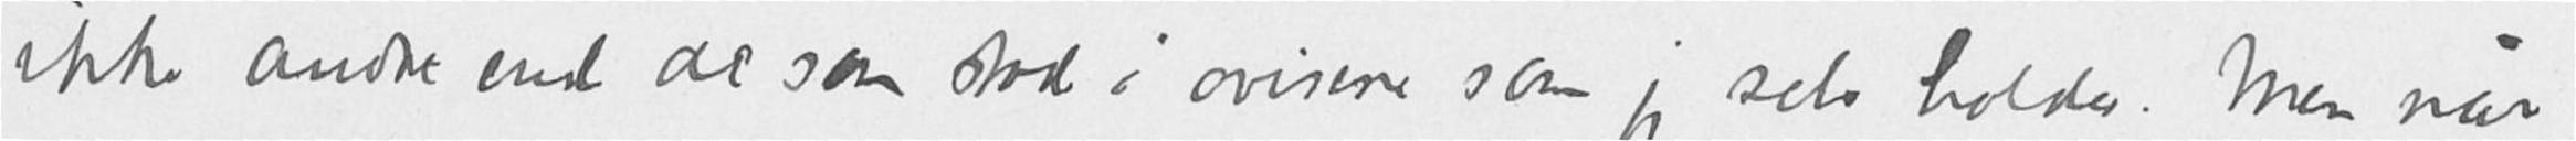ikke andre end de som stod i avisene som jeg selv holder. Men når
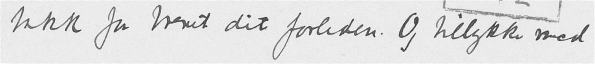takk for brevet dit forleden. Og tillykke med
